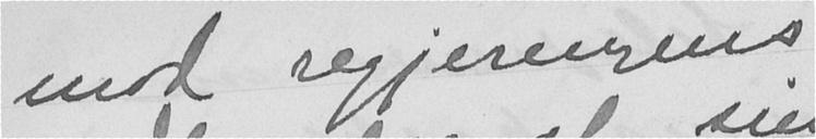mod regjeringens
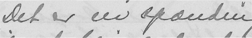Det er en spænding
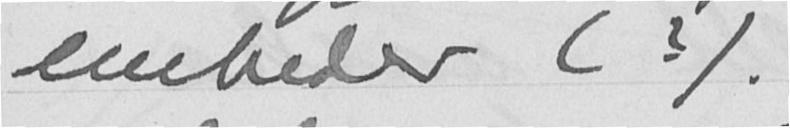embeder (?).
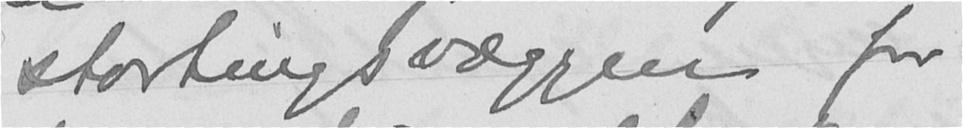stortingsvæggen for
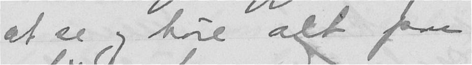at se og høre alt paa
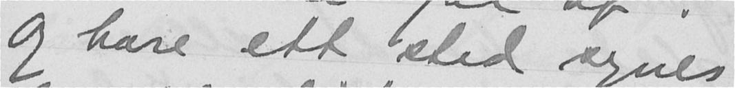Og bare ett sted synes
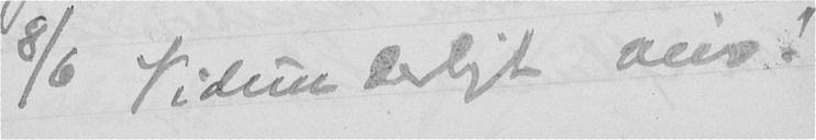8/6 Vidunderligt veir!
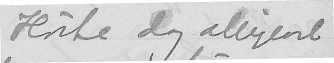Hørte dog alligevel
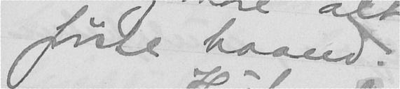første haand.
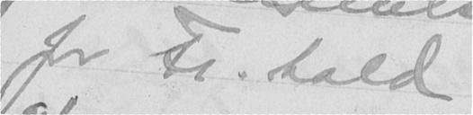for Fr. hald.
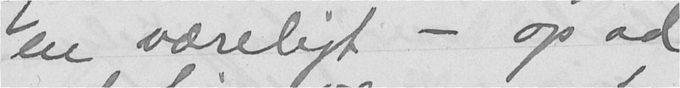en væreligt - op ad
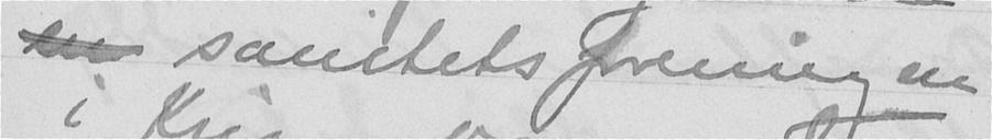en sanitetsforeningen
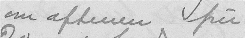om aftenen fru
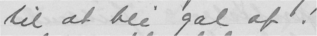til at bli gal af!
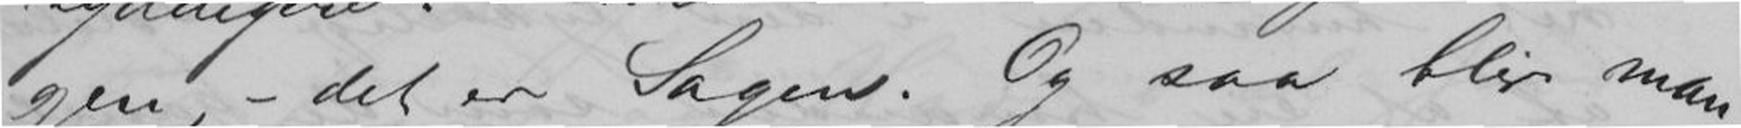gen, - det er Sagen. Og saa blir man
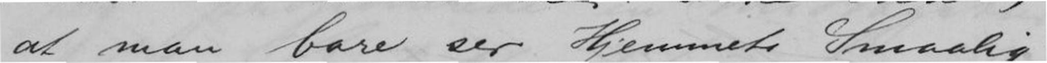at man bare ser Hjemmets Smaalig-
